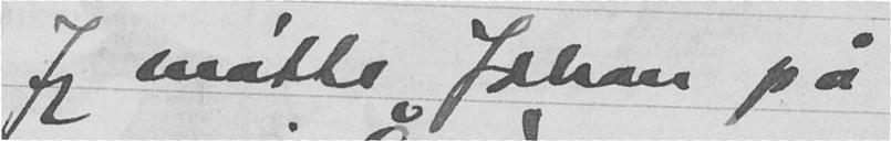Jeg møtte Johan på
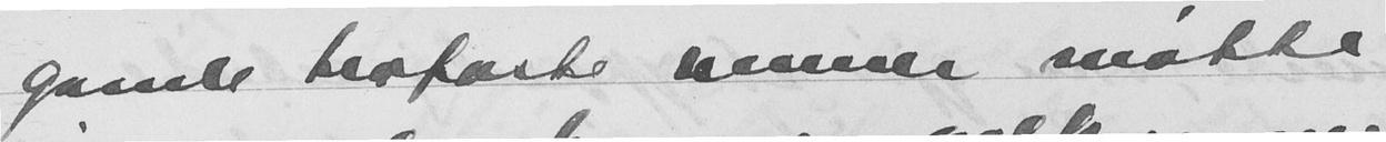gamle trofaste venner møtte
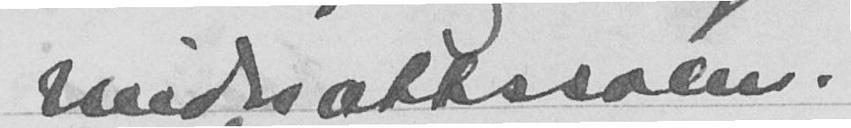midnattssolen.
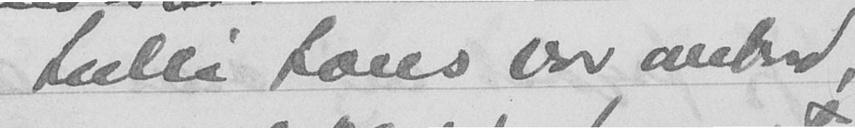Tretti fans var ombord
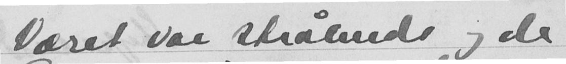Været var strålende og de
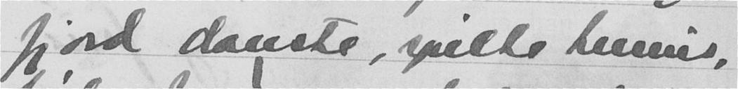fjord danste, spilte tennis,
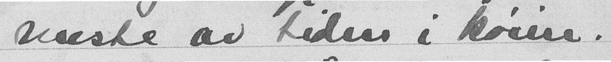meste av tiden i køien.
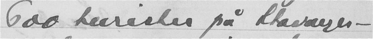600 turister på Stavanger-
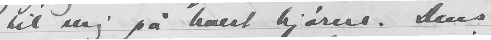til mig på hvert hjørne. Dens
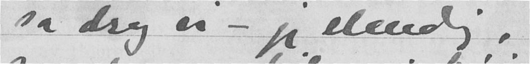så drog vi - jeg elendig,
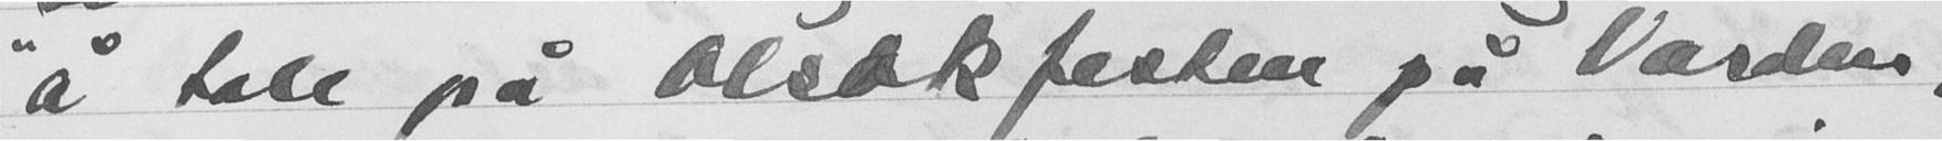å tale på Olsokfesten på Varden
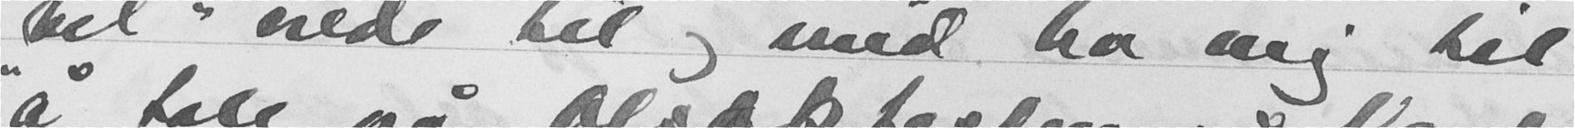"vel" vilde til og med ha mig til
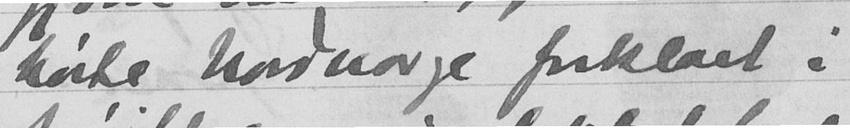hørte Nordnorge forklart i
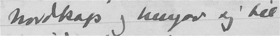Nordkap og hengav sig til
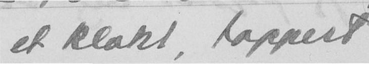et klokt tappert
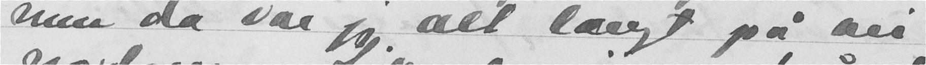men da var jeg alt langt på vei
